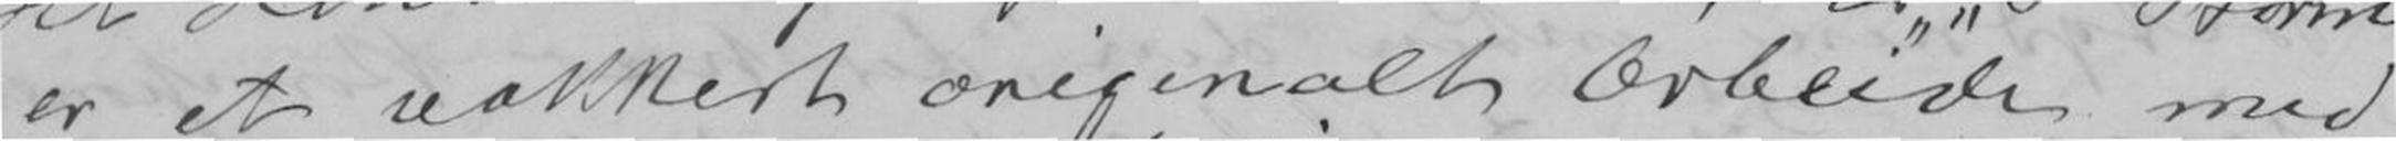er et vakkert originalt Arbeide med
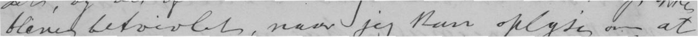blive betvivlet, naar jeg kan oplyse om at
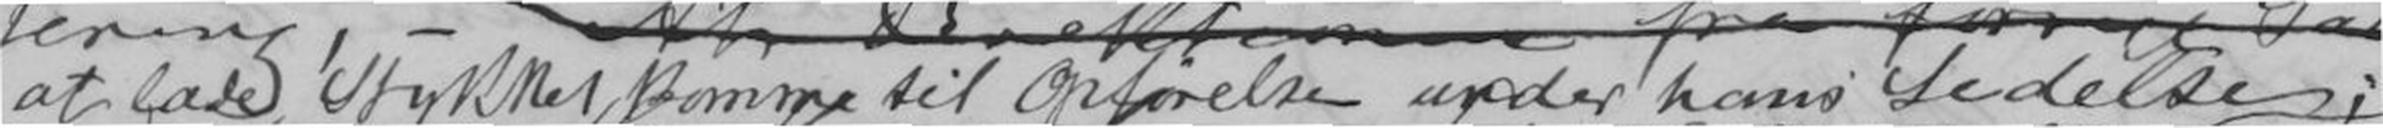at lade Stykket komme til Opførelse under hans Ledelse;
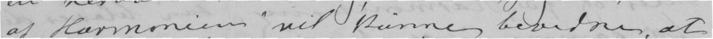af Harmonien vil kunne bevidne, at
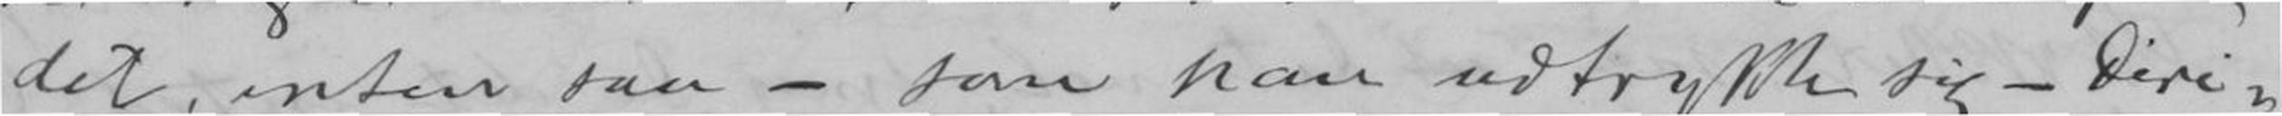det, enten saa - som han udtrykke sig - Dire-
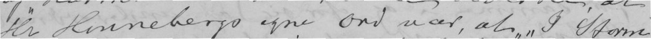Hr Hennebergs egne Ord var, at "I Storm
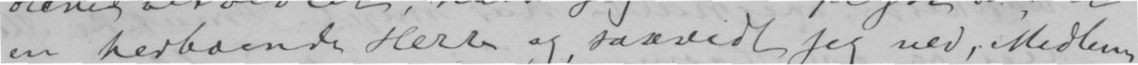en herboende Herre og saavidt jeg ved, Medlem
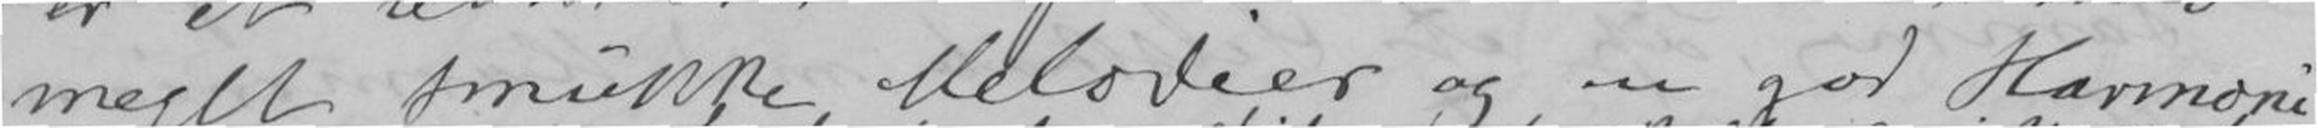meget smukke Melodier og en god Harmoni-
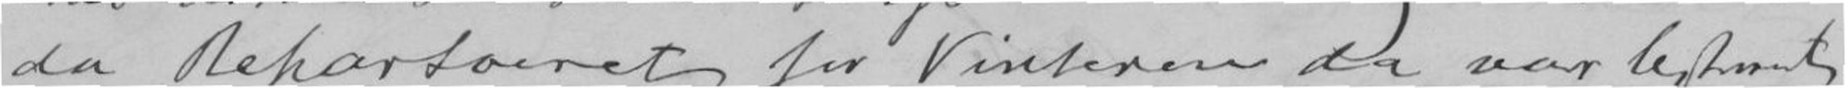da Reportoaret for Vinteren da var bestemt
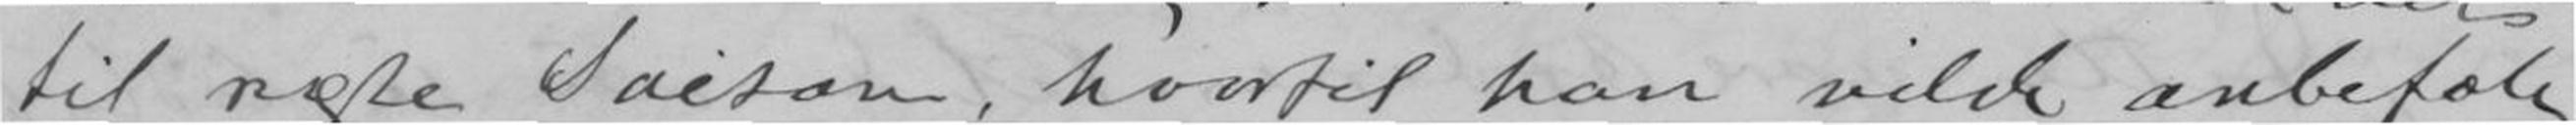til næste Saison, hvortil han vilde anbefale
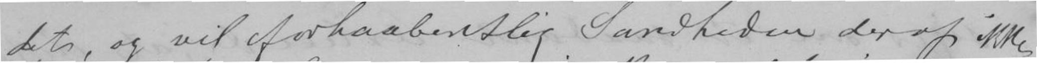det, og vil forhaabentlig Sandheden deraf ikke
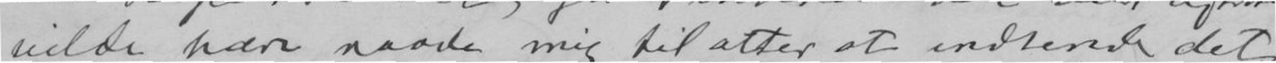vilde han raade mig til atter at indsende det
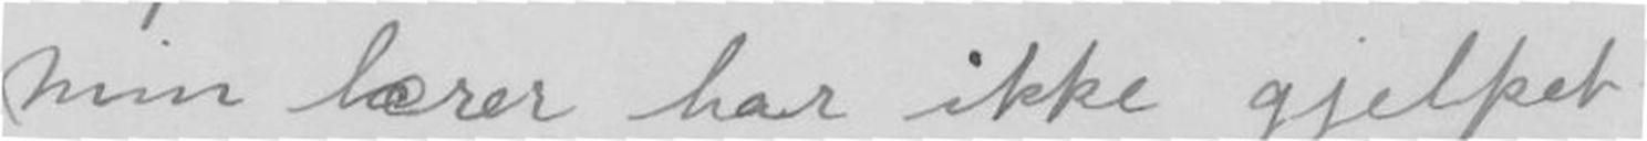Min lærer har ikke gjelpet
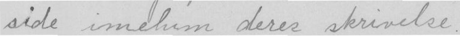side imelum deres skrivelse.
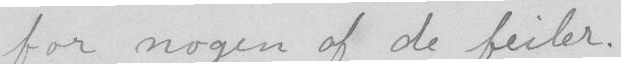for nogen af de feiler.
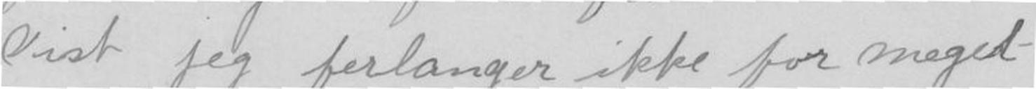Vist jeg ferlanger ikke for meget
